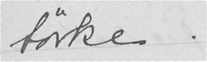tørke.
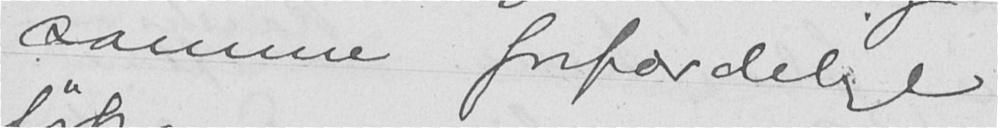samme forfærdelige
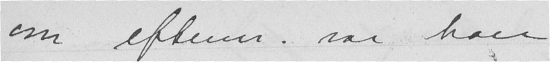om efterm. var han
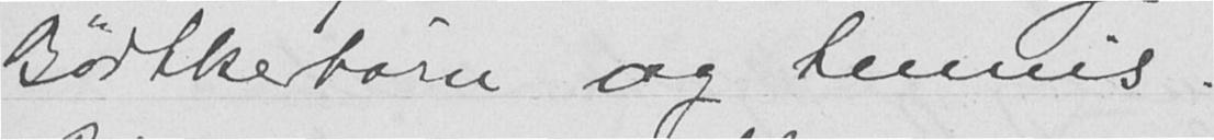Bødtkerbørn og tennis.
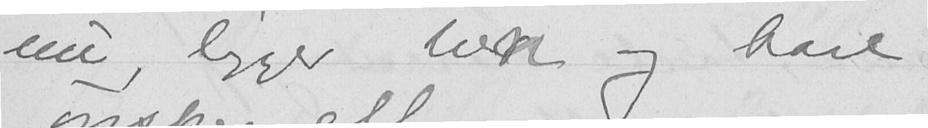nu, ligger hen og bare
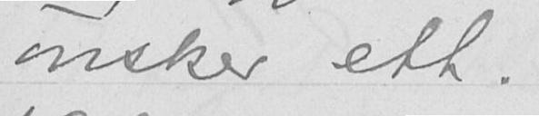ønsker ett.
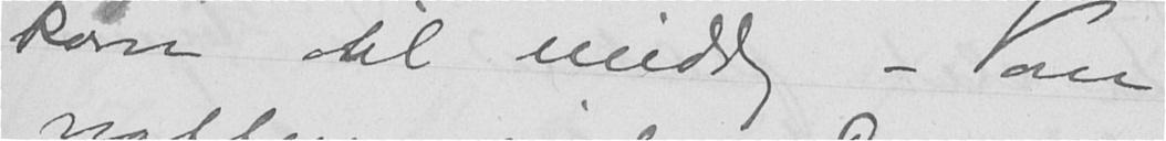kom til middag - om
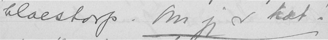Claestorp. Om jeg er træt!
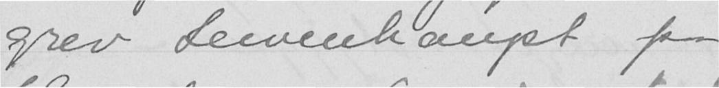grev Lewenhaupt paa
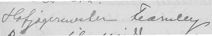Hofjægermester Fearnleys
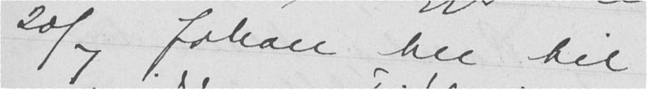20/7 Johan her til
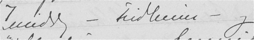middag - Fridheim - og
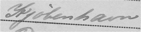Kjøbenhavn
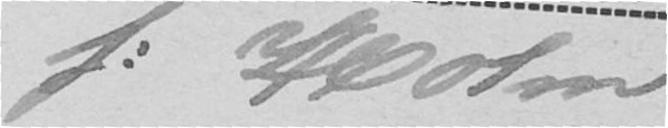f. Holm
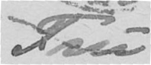Fru
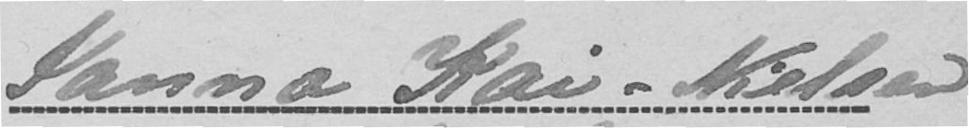Janna Kai-Nielsen
